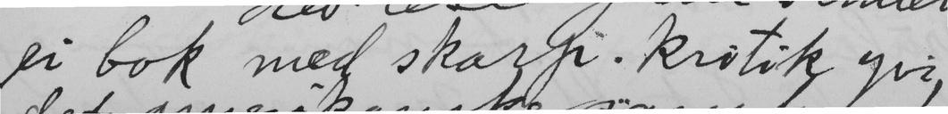ei bok med skarp kritik yvi
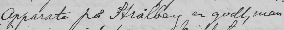Apparate på Strålberg er godt, men
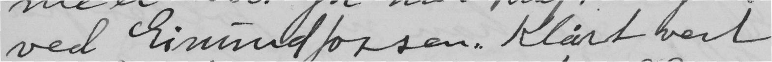ved Eimundfossen. Klårt vert
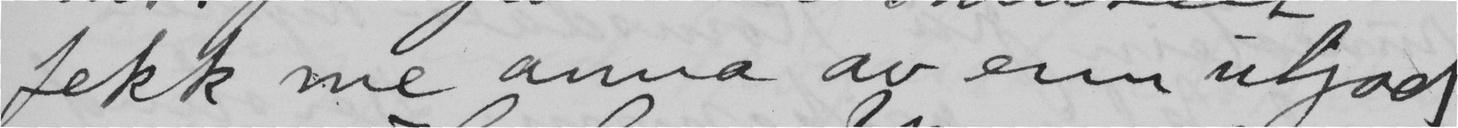fekk me anna av enn uljod.
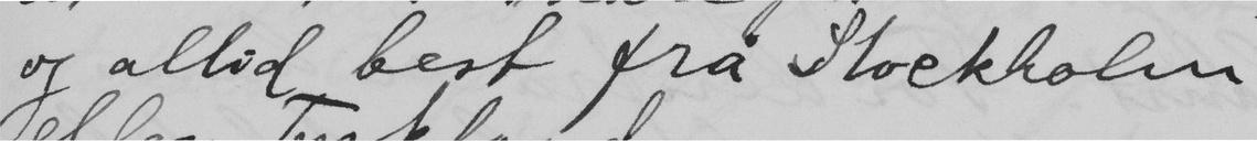og altid best fra Stockholm
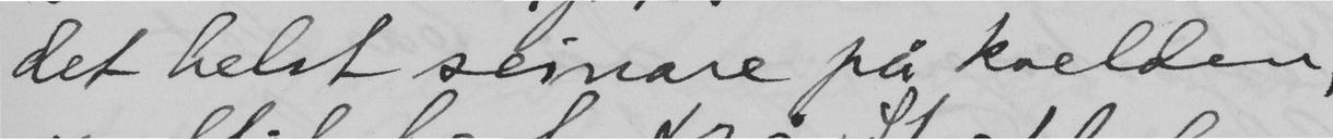det helst seinare på kvelden,
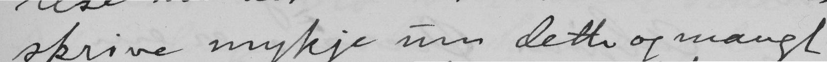skrive mykje um dette og mangt
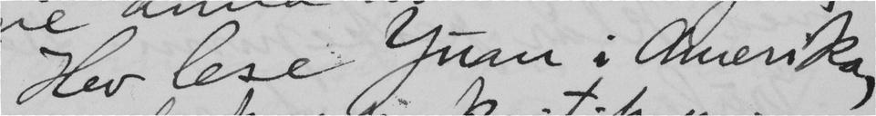Hev lese Juan i Amerika,
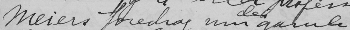Meiers foredrag um dei gamle
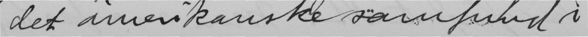det amerikanske samfund i
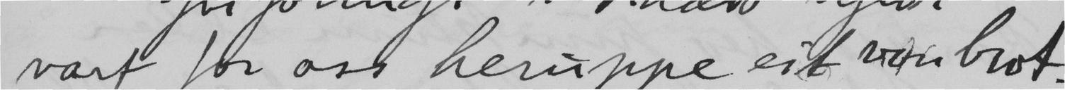vart for oss heruppe eit vonbrot.
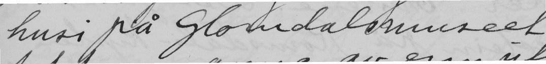husi på Glomdalsmuseet
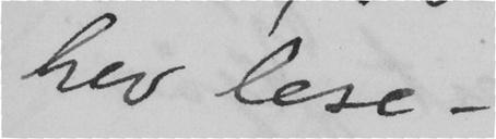hev lese –
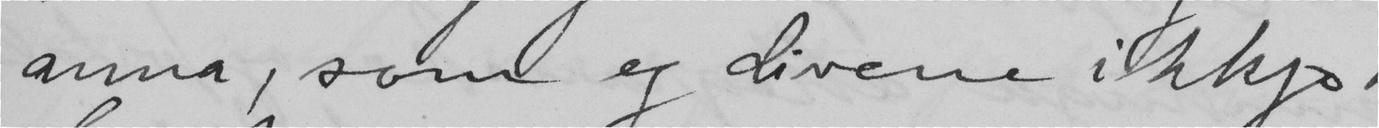anna, som eg diverre ikkje
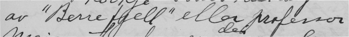av "Berre fjell" eller professor
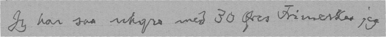Jeg har saa uhyre med 30 Øres Frimerker jeg
